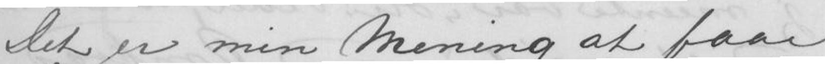Det er min Mening at faae
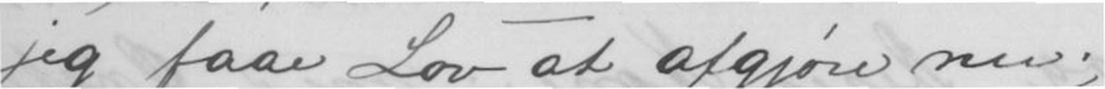jeg faae Lov at afgjøre nu;
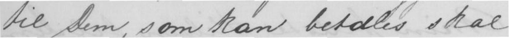til Dem, som kan betales skal
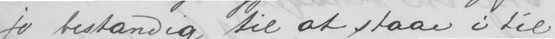jo bestandig til at staae i til
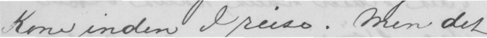Kone inden De reise. Men det
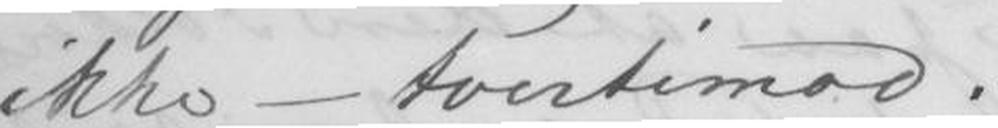ikke - tvertimod.
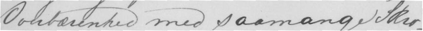Overbærenhed med saamange Skrø-
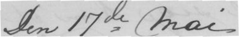Den 17de Mai.
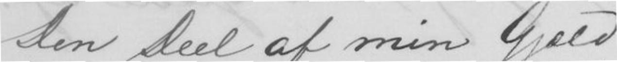Den Deel af min Gjæld
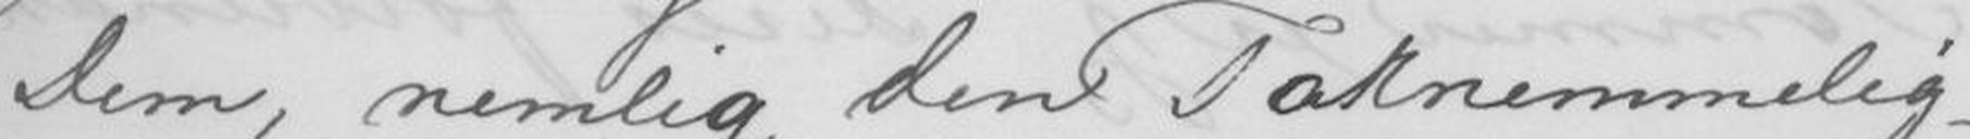Dem, nemlig den Taknemmelig-
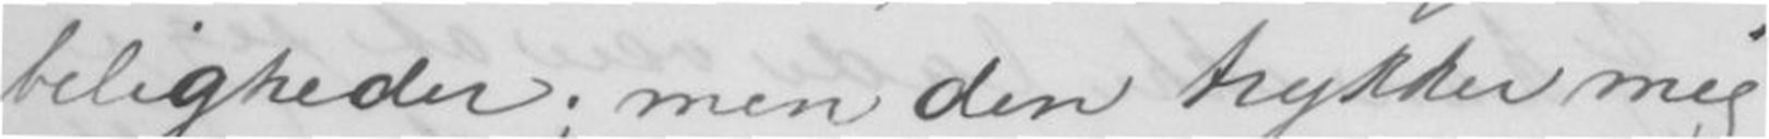beligheder; men den trykker mig
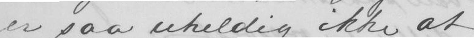er saa uheldig ikke at
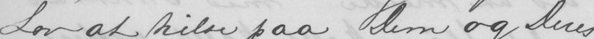Lov at hilse paa Dem og Deres
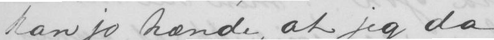kan jo hænde, at jeg da
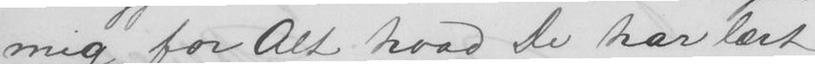mig for Alt hvad De har lært
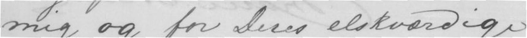mig og for Deres elskværdige
